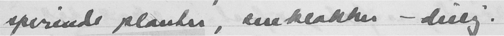spirinde planter, sneklokker - deilig: .
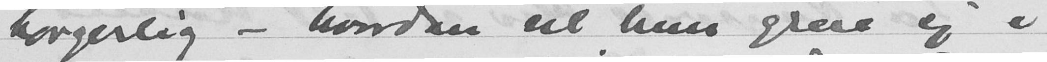borgerlig - hvordan vil hun greie seg i
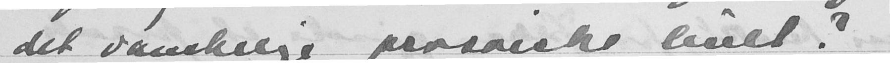det vanskelige prosaiske livet?
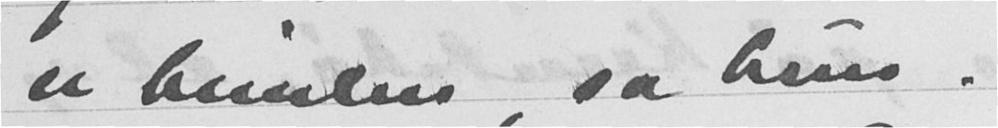er himlen sa hun.
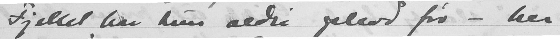Fjellet har hun aldri oplevd før - her
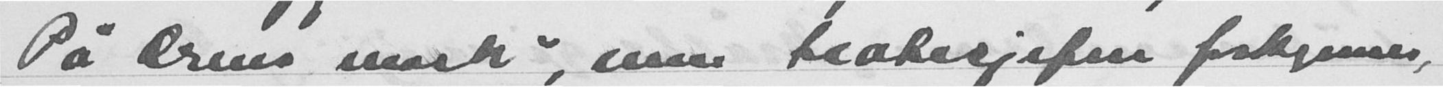"På ærens mark", men teatersjefen forkynner
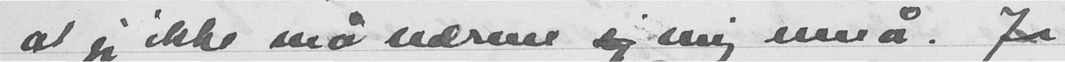at jeg ikke må nærme  mig ennå. Ja
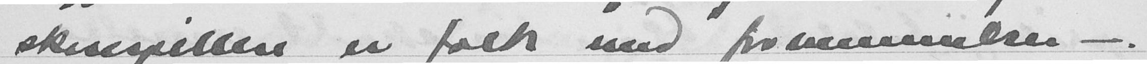skuespillere er folk med formenkser -.
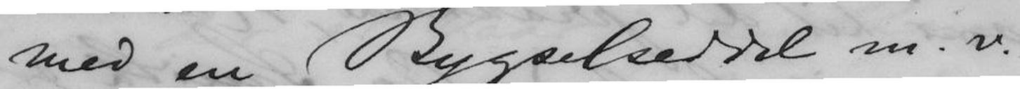med en Bygselseddel m.v. -
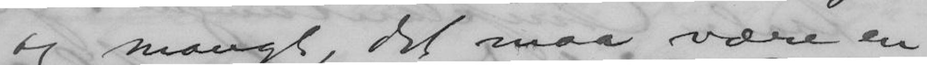og mangt, det maa være en
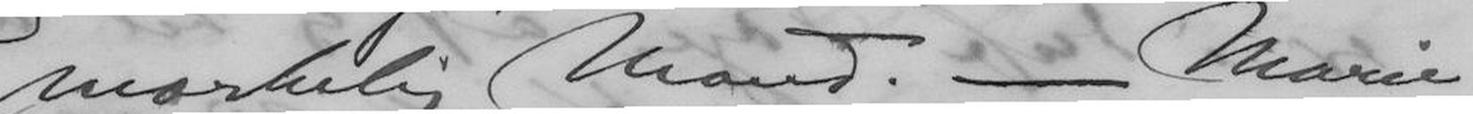mærkelig Mand. - Marie
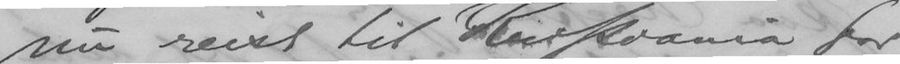nu reist til Kristiania for
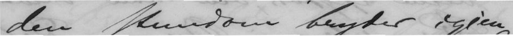den stundom bryder igjen-
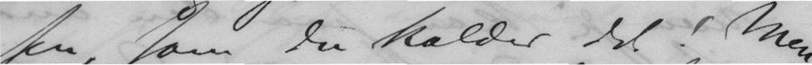sen, som Du kalder det! Men
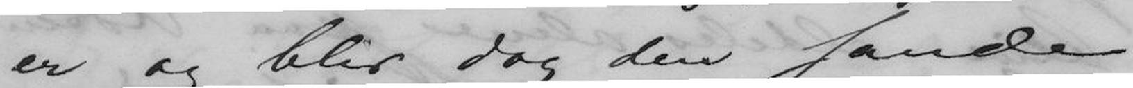er og blir dog den sande
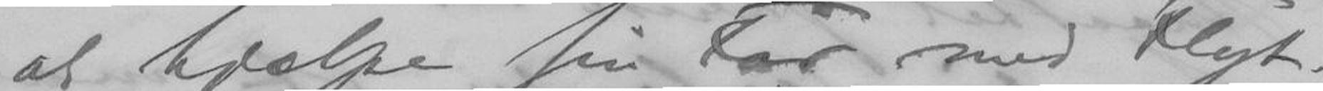at hjælpe sin Far med Flyt-
